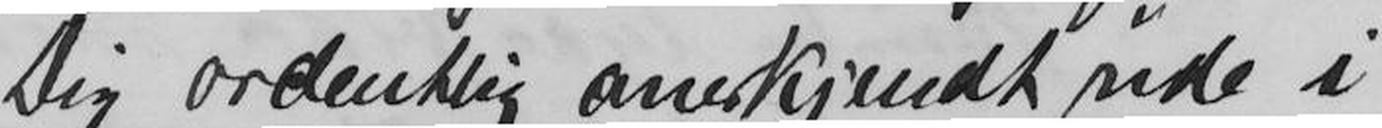Dig ordentlig anerkjendt ude i
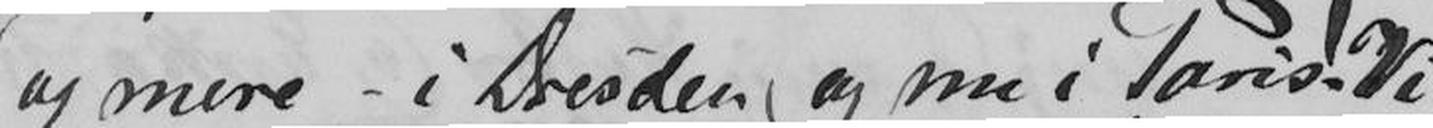og mere - i Dresden og nu i Paris! Vi
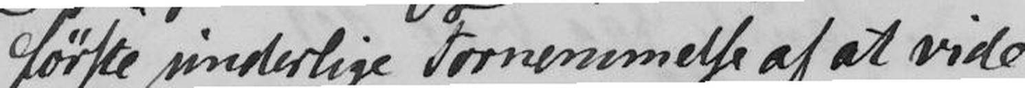første inderlige Fornemmelse af at vide
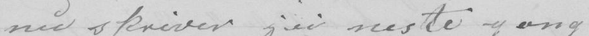nu skriver jei neste gang
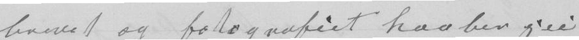brevet og fotografiet haaber jei
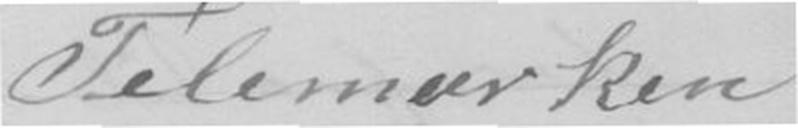Telemarken
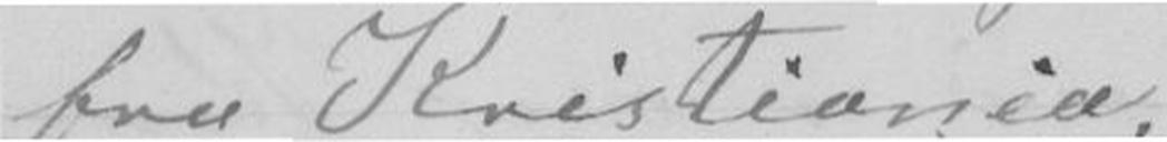fra Kristiania,
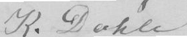K. Dahle
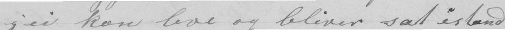jei kan leve og bliver sat istand
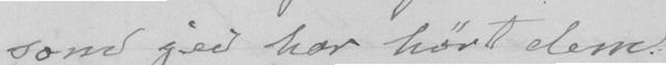som jei har hørt dem.
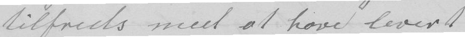tilfreds med at hove levert
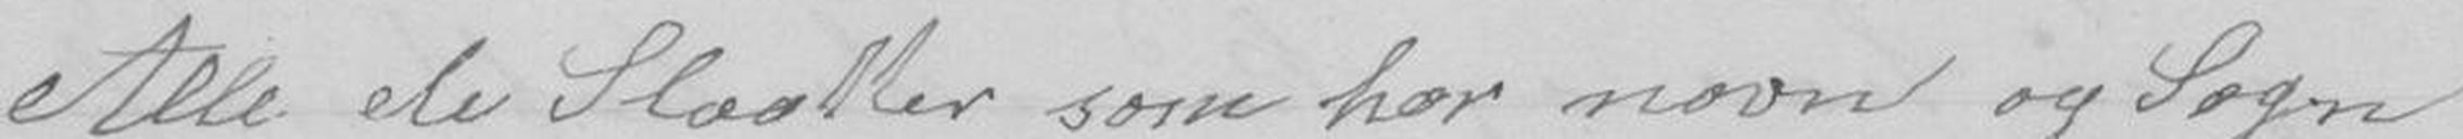Alle de Slaatter som har navn og Sagn
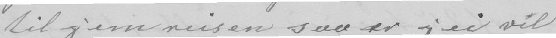til jem reisen saa er jei vel
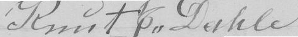Knut J. Dahle
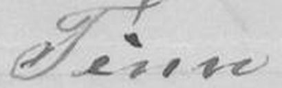Tinn
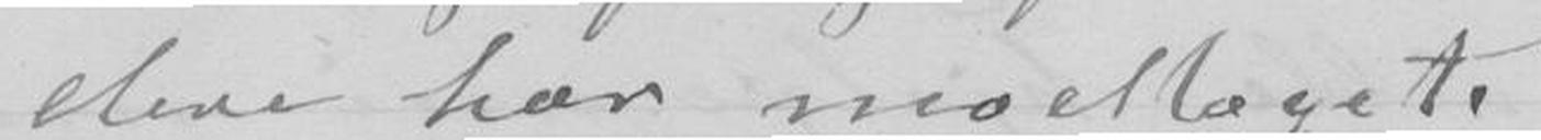dere har modtaget.
